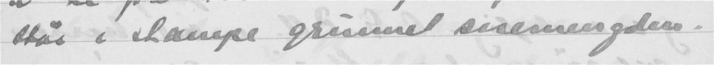står i stampe grunnet snemengden.
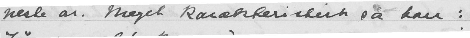neste år. Meget karakteristisk sa han:
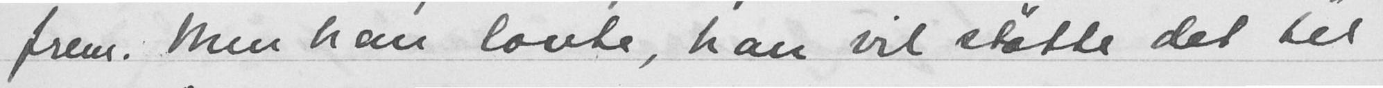frem. Men han lovte, han vil støtte det til
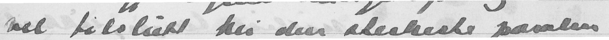vel tilslutt bli den sterkeste panten
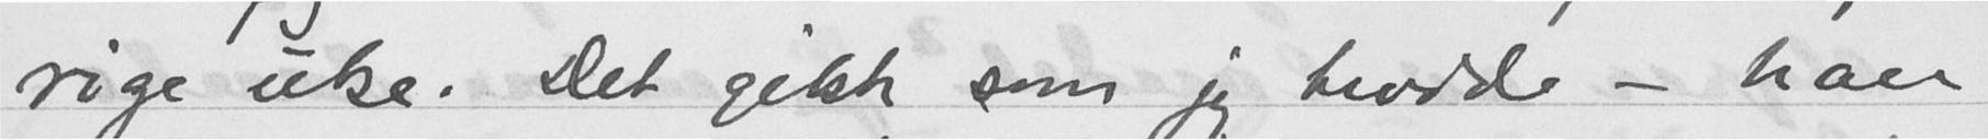rige uke. Det gikk som jeg trodde - han
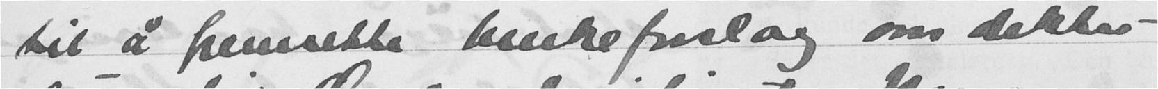til å fremsette benkeforslag om dikter-
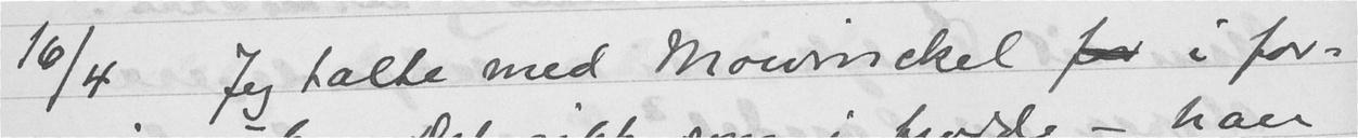16/4 Jeg talte med Mowinckel  i for-
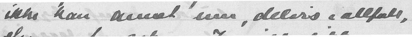ikke kan annet enn, delvis i allfall,
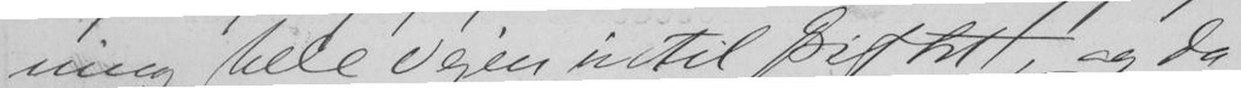ning hele vejen intil skiftet, og da
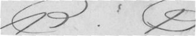B. B.
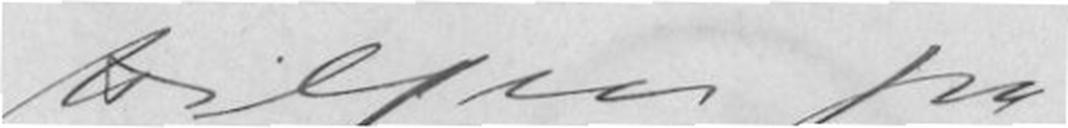Hilsen fra
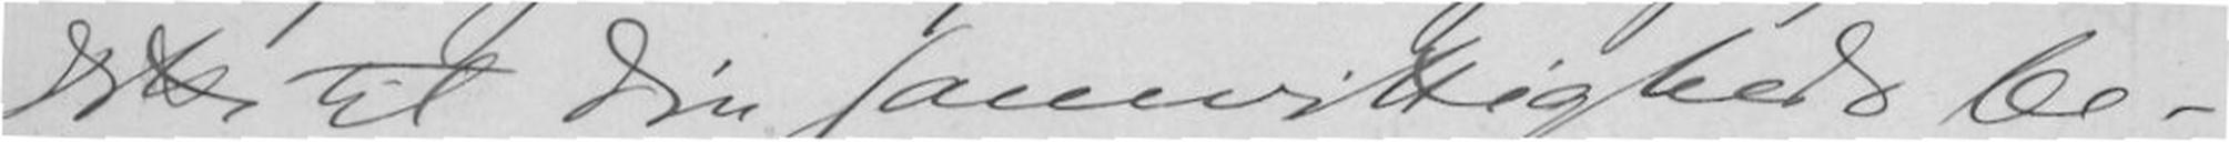Dette til din samvittigheds be-
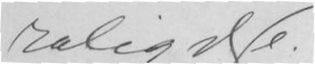roligelse.
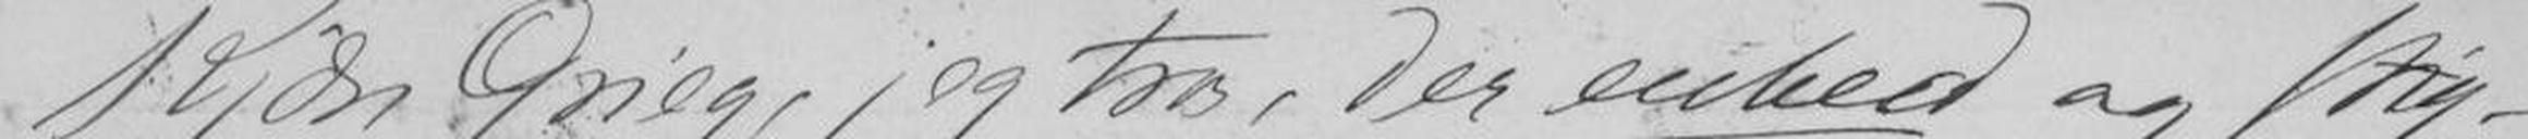Kjære Grieg, jeg tror, der enhed og stig-
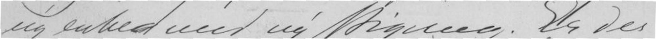ny enhed med ny stigning. Er der
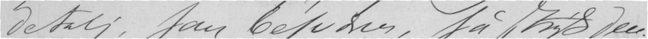detalj, som besværer, så stryk den.
